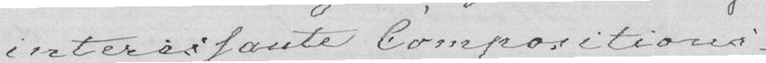interessante Compositions-
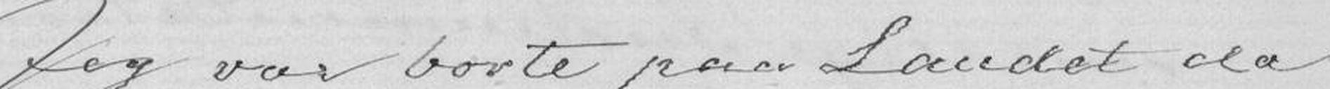Jeg var borte paa Landet da
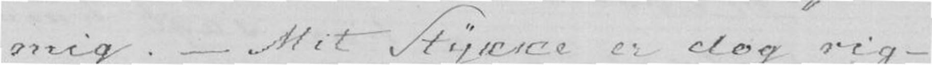mig.- Mit Stykke er dog rig-
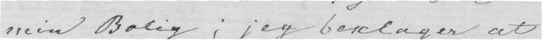min Bolig; jeg beklager at
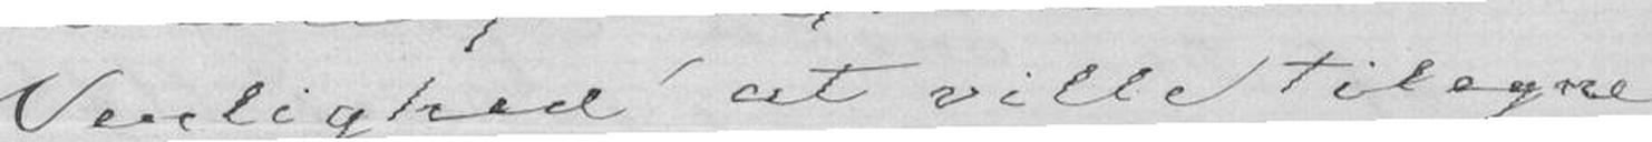Venlighed at ville tilegne
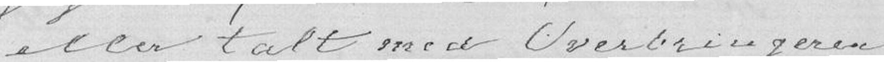eller talt med Overbringeren
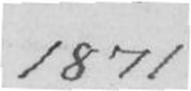1871
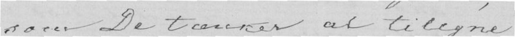som De tænker at tilegne
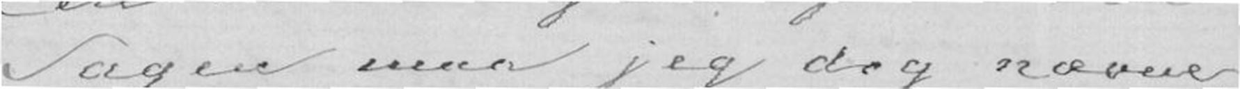Sagene maa jeg dog nævne,
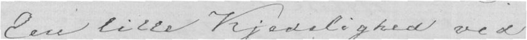Een lille Kjedelighed ved
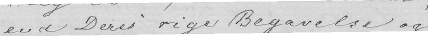end Deres rige Begavelse og
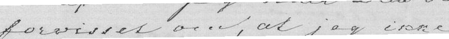forvisset om, at jeg ikke
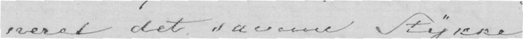neret det samme Stykke,
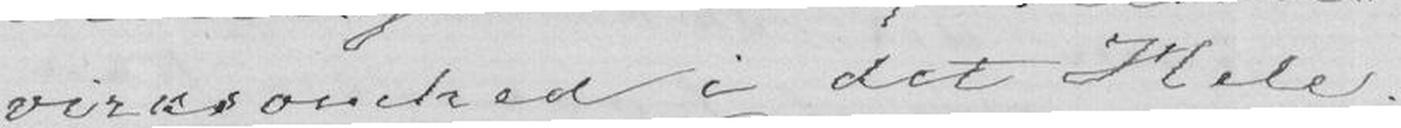virksomhed i det Hele.
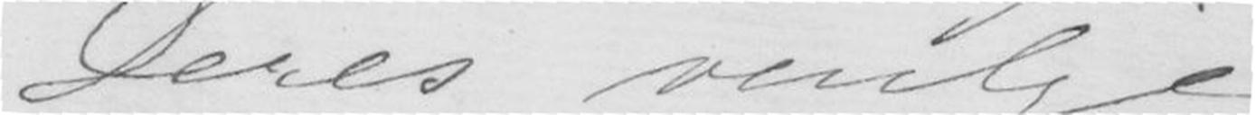Deres venlige
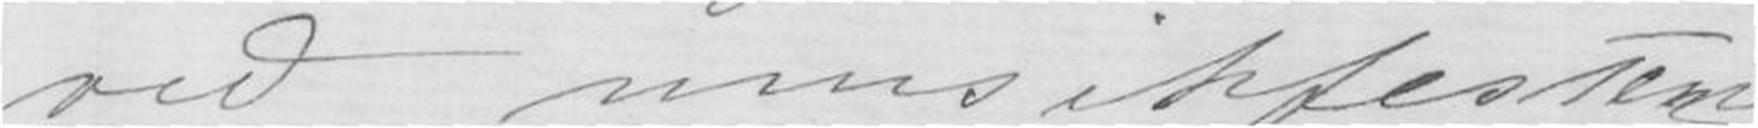ved musikfesten
